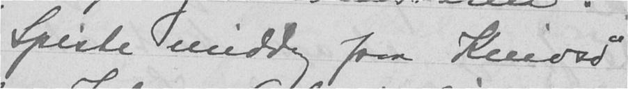Spiste middag paa Knivsø
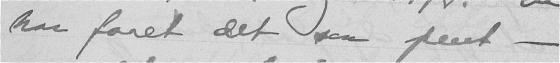har faaet det saa pent -
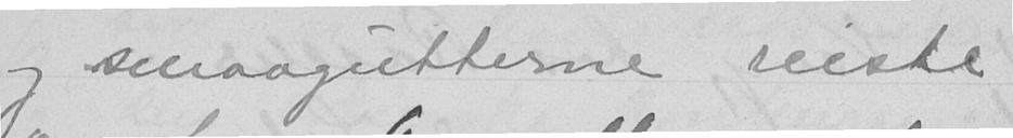og smaagutterne reiste
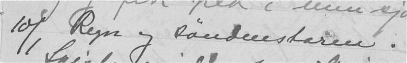10/1 Regn og søndenstorm.
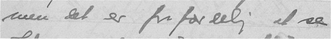men det er forfærdelig at se
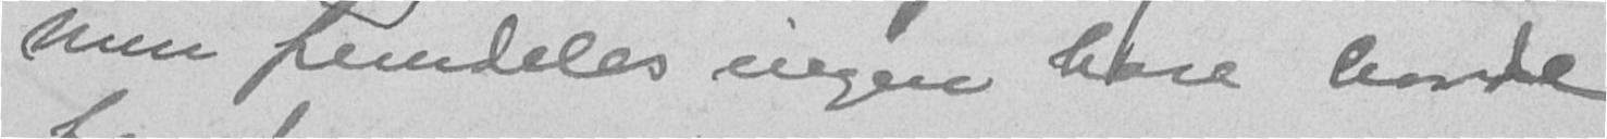men fremdeles ingen hare havde
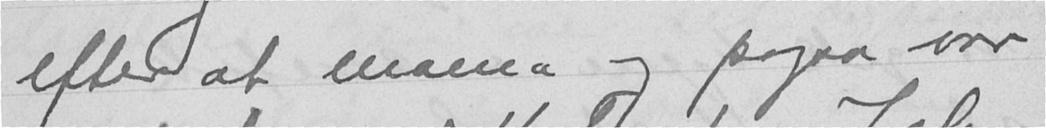efter at mama og papa var
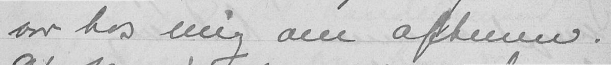var hos mig om aftenen.
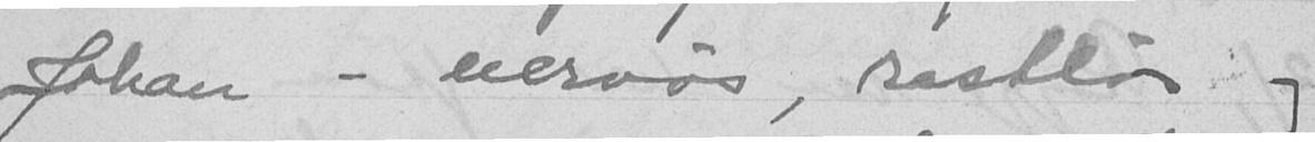Johan - nervøs, rastløs og
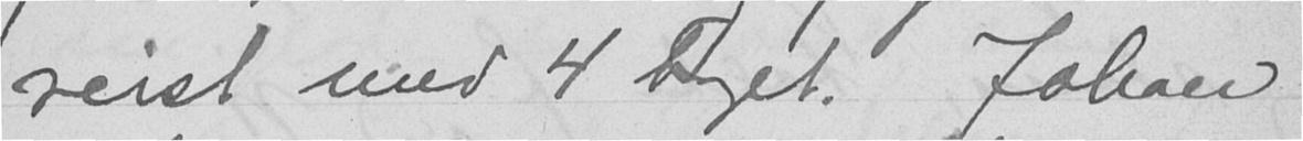reist med 4 toget. Johan
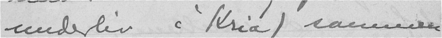underliv i Kria) sammen
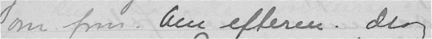om form. Om efterm. drog
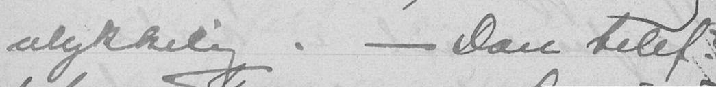ulykkelig. - Don telef.
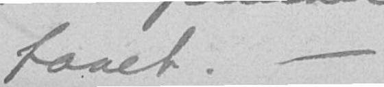faaet. -
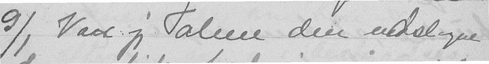9/1 Var jeg alene den nedslagne
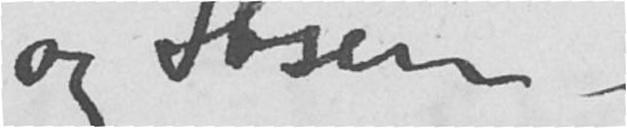og Ibsen. -
